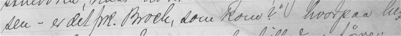sen - er det frk. Broch, som kom?" hvorpaa hu
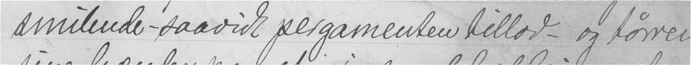smilende - saavidt pergamenten tillod - og tørre
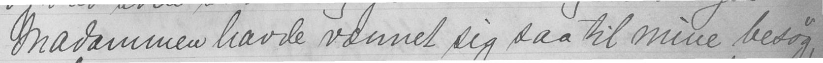Madammen havde vænnet sig saa til mine besøg,
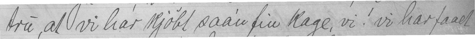tru, at vi har kjøbt saa'n fin kage, vi! vi har faaet
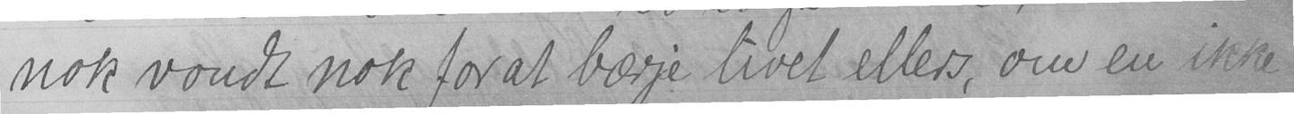nok vondt nok for at bærje livet ellers, om en ikke
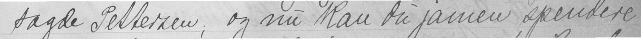sagde Pettersen; og nu kan du jamen spendere
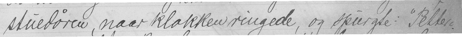stuedøren, naar klokken ringede, og spurgte: "Petter-
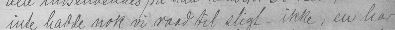inte hadde nok vi raad til sligt - ikke; en har
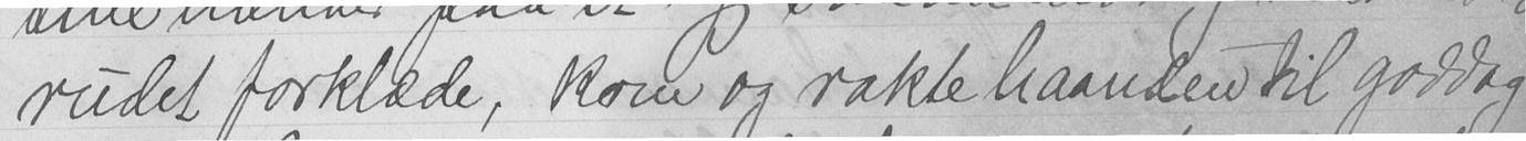rudet forklæde, kom og rakte haanden til goddag
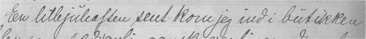En lillejuleaften sent kom jeg ind i butikken
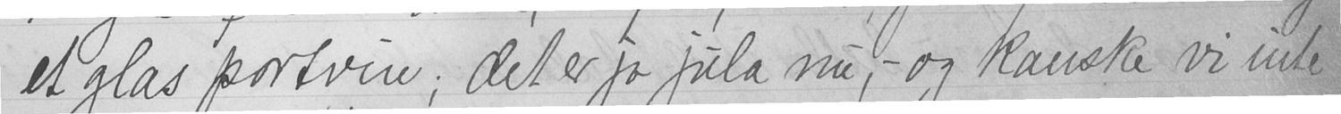et glas portvin; det er jo jula nu, - og kanske vi inte
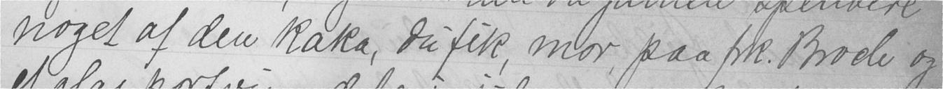noget af den kaka, du fik, mor, paa frk. Broch og
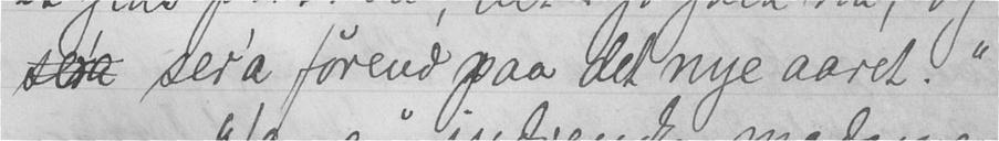ser'a ser'a førend paa det nye aaret."
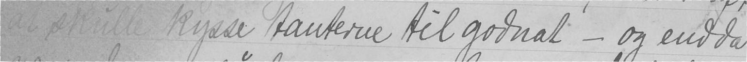at skulle kysse tanterne til godnat - og endda
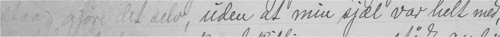staa gjøre det selv, uden at min sjæl var helt med,
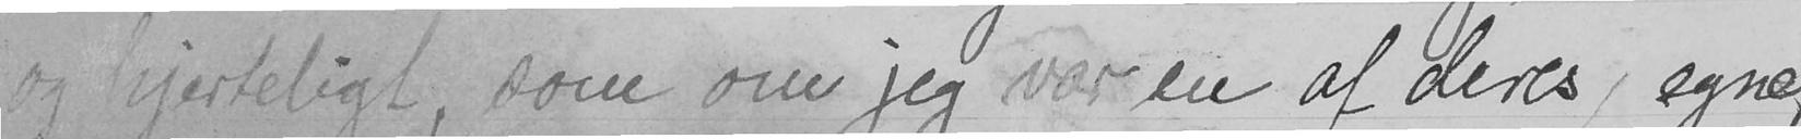og hjerteligt, som om jeg var en af deres egne,
